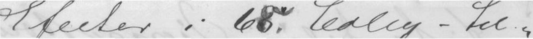Efecter i 68 Colly - til-
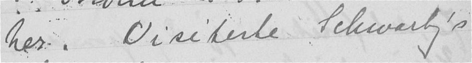her. Visiterte Schwartz's
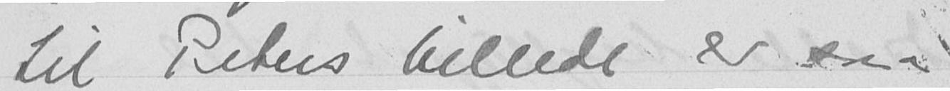til Peters billede er saa
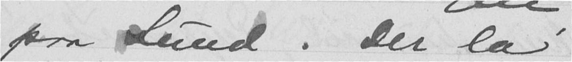paa Sund. Der la'
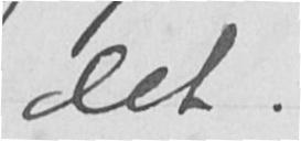det.
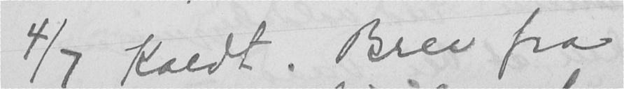4/7 Koldt. Brev fra
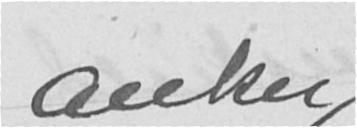Anker
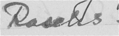Raschs.
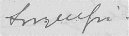Sorgenfri.
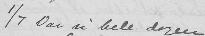1/7 Var vi hele dagen
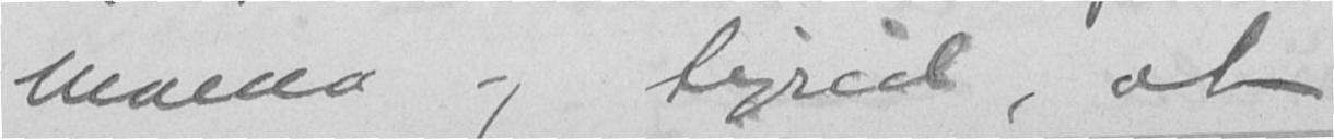mama og Sigrid, at
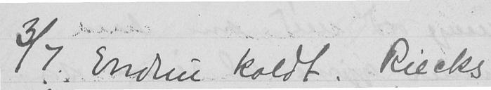3/7. Endnu koldt. Riecks
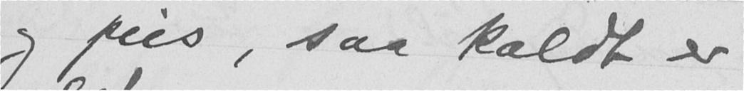og peis, saa koldt er
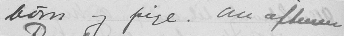børn og pige. Om aftenen
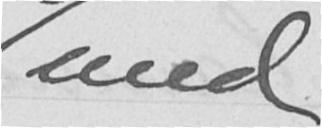med
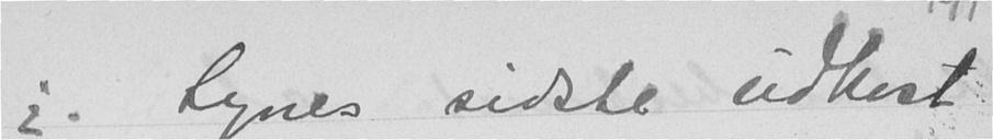i. Synes sidste udkast
